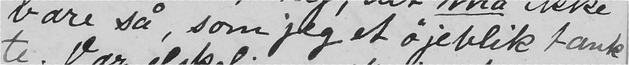være så, som jeg et øjeblik tænk-
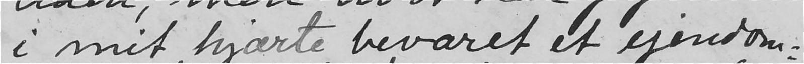i mit hjærte bevaret et egendom-
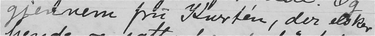gjennem fru Kurtén, der elsker
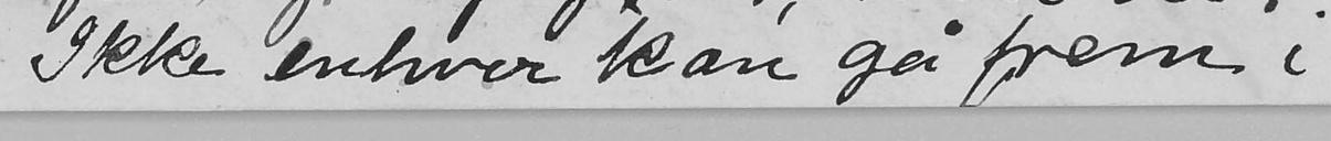Ikke enhver kan gå frem i
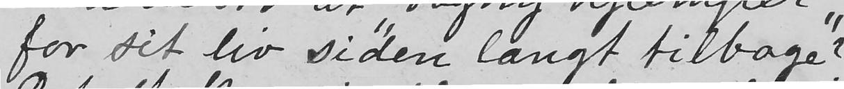for sit liv siden langt tilbage"?
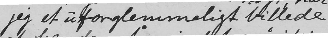jeg et uforglemmeligt billede
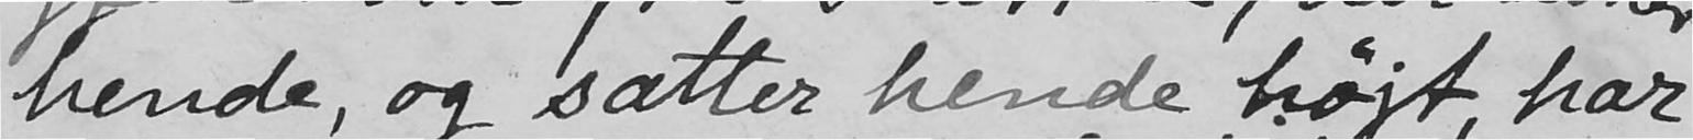hende, og sætter hende højt har
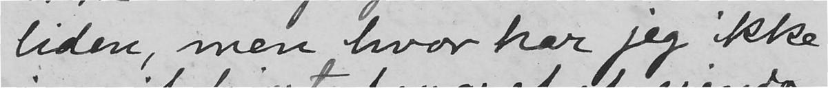liden, men hvor har jeg ikke
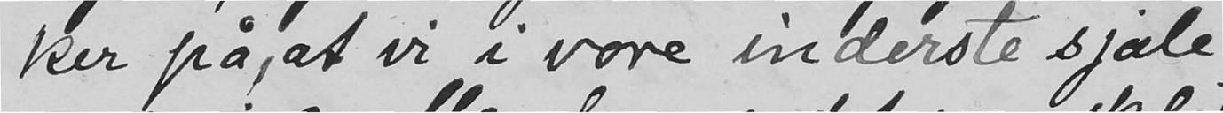ker på at vi i vore inderste sjæle
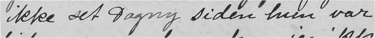ikke set Dagny siden hun var
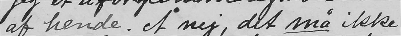af hende. Å nej, det må ikke
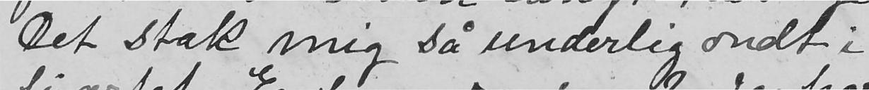Det stak mig så underlig ondt i
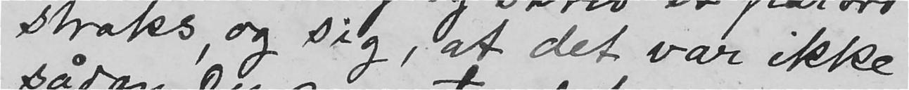straks, og sig, at det var ikke
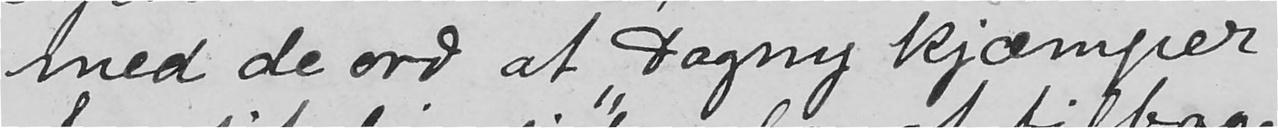med de ord at "Dagny kjæmper
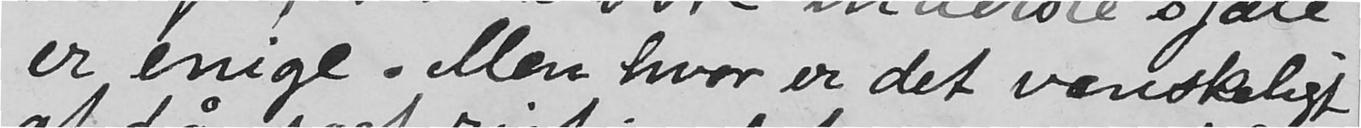er enige. Men hvor er det vanskeligt
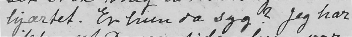hjærtet. Er hun da syg? Jeg har
